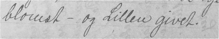blomst - og Lillen givet.
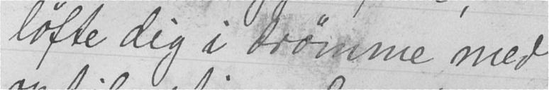løfte dig i drømme med
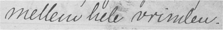mellem hele vrimlen.
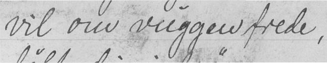vil om vuggen frede,
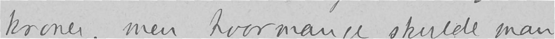kroner, men hvormange skulde man
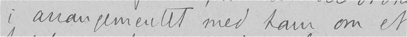i arangementet med ham om et
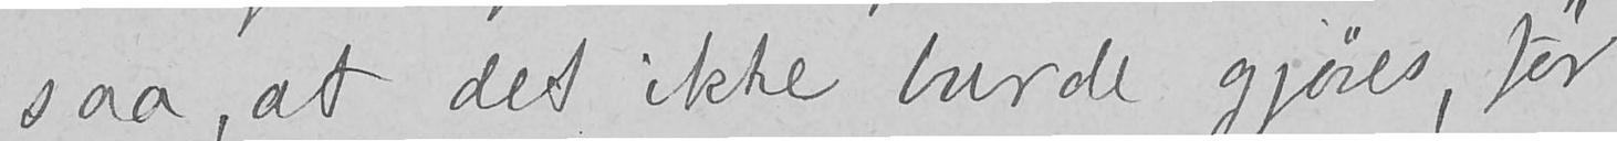saa at det ikke burde gjøres, før
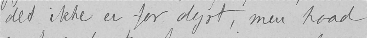det ikke er for dyrt, men hvad
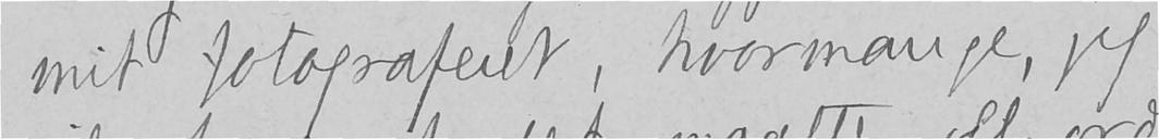mit fotograferet, hvormange, jeg
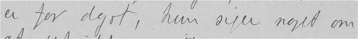er for dyrt, hun siger noget om,
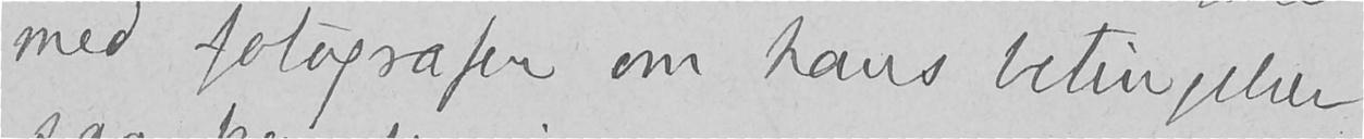med fotografen om hans betingelser
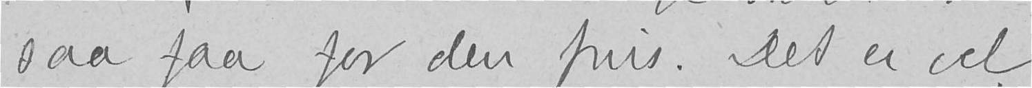saa faa for den pris. Det er vel
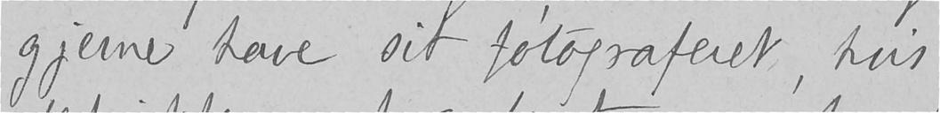gjerne have sit fotograferet, hvis
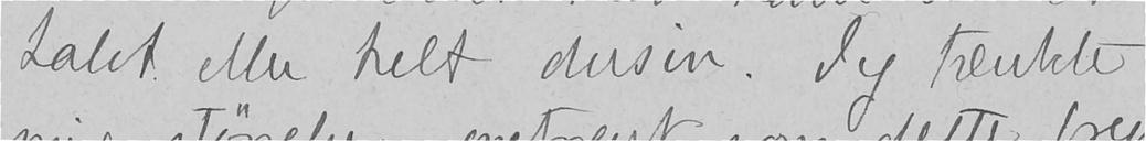halvt eller helt dusen. Jeg tænkte
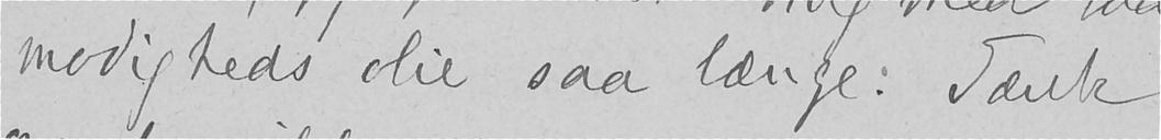modigheds olie saa længe. Tænk
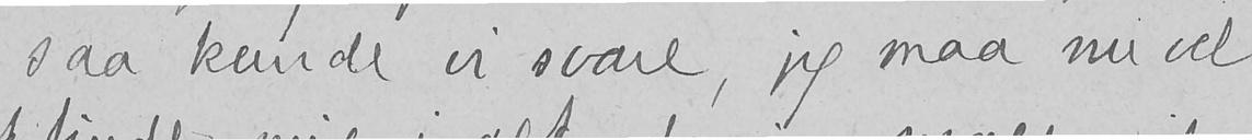saa kunde vi svare, jeg maa nu vel
